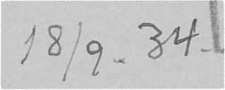18/9. 34.
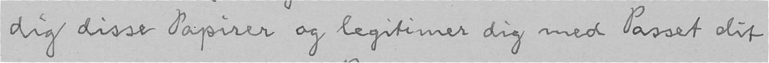dig disse Papirer og legitimer dig med Passet dit
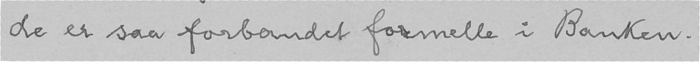de er saa forbandet formelle i Banken.
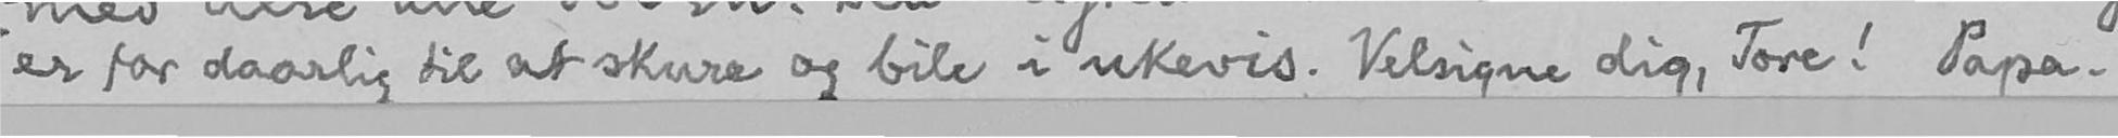er for daarlig til at skure og bile i ukevis. Velsigne dig, Tore! Papa.
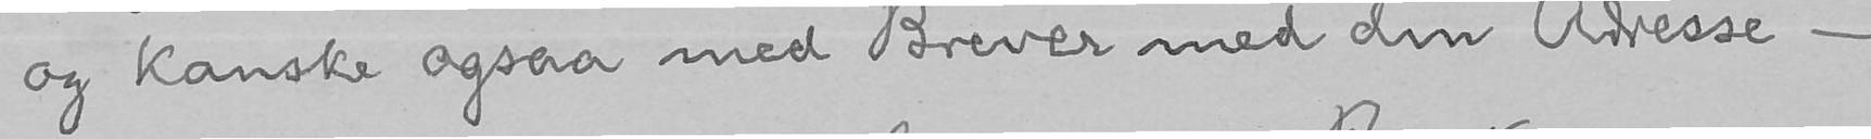og kanske ogsaa med Brever med din Adresse -
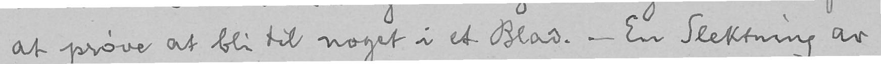at prøve at bli til noget i et Blad. - En Slektning av
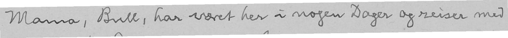Mama, Bull, har været her i nogen Dager og reiser med
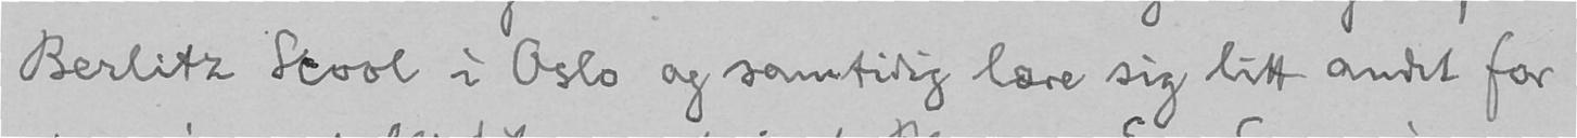Berlitz Scool i Oslo og samtidig lære sig litt andet for
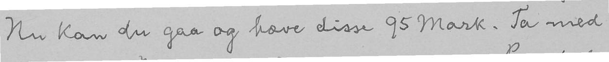Nu kan du gaa og hæve disse 95 Mark. Ta med
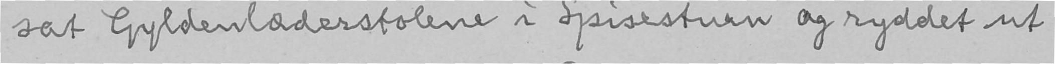sat Gyldenlæderstolene i Spisestuen og ryddet ut
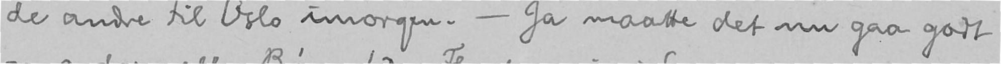de andre til Oslo imorgen. - Ja maatte det nu gaa godt
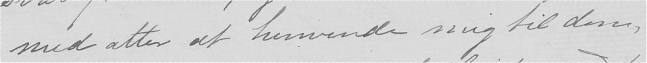med atter at henvende mig til dem,
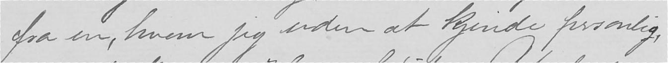fra en, hvem jeg uden at kjende personlig,
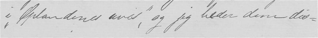i  "Oplandenes avis", og jeg beder dem dis-
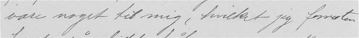være noget til mig, hvilket jeg forresten
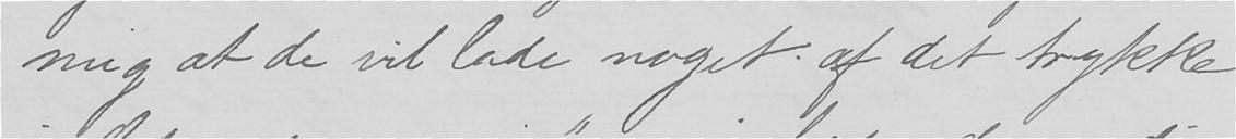mig at de vil lade noget af det trykke
